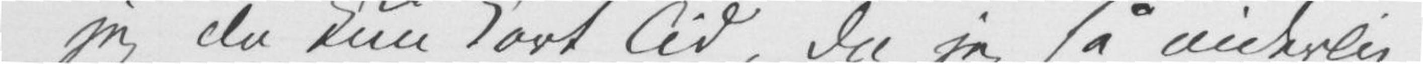jeg da kun kort Tid, da jeg så inderlig
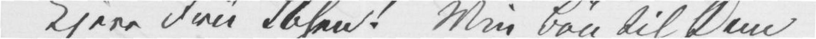Kjere Fru Ibsen! Min Bøn til Dem
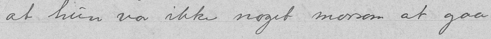at hun var ikke noget morsom at gaa
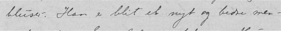bluser –. Han er blit et nyt og bedre men-
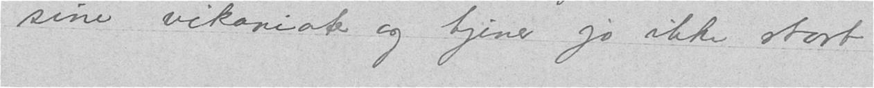sine vikariater og tjener jo ikke stort –
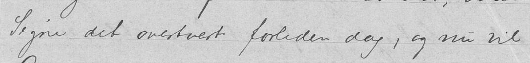Signe det overtvert forleden dag, og nu vil
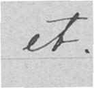et.
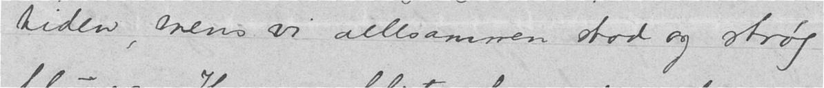tiden, mens vi allesammen stod og strøg
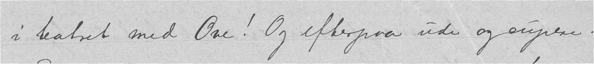i teatret med Ove! Og efterpaa ude og supere.
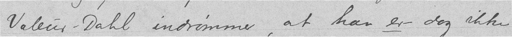Valeur-Dahl indrømmer, at han er dog ikke
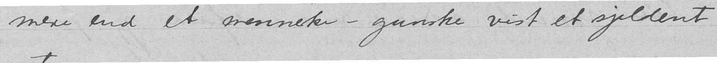mere end et menneke – ganske vist et sjeldent
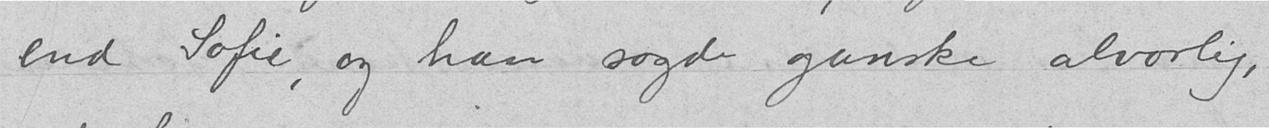end Sofie, og han sagde ganske alvorlig,
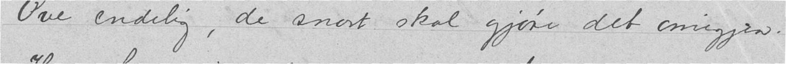Ove endelig, de snart skal gjøre det omigjen.
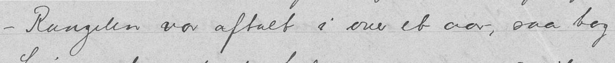– Rangelen var aftalt i over et aar, saa tog
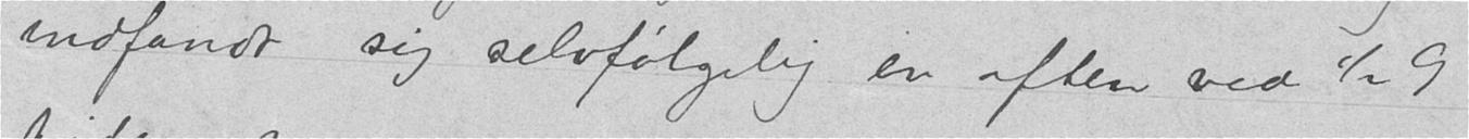indfandt sig selvfølgelig en aften ved ½ 9
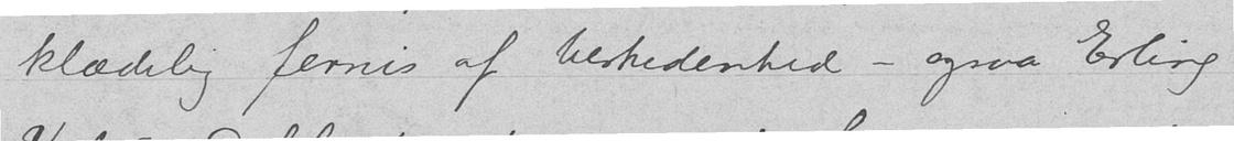klædelig fernis af beskedenhed – ogsaa Erling
